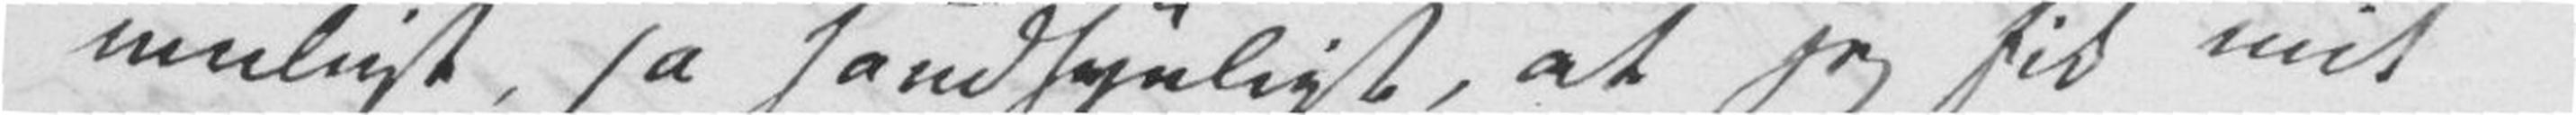muligt, ja sandsynligt, at jeg fik mit
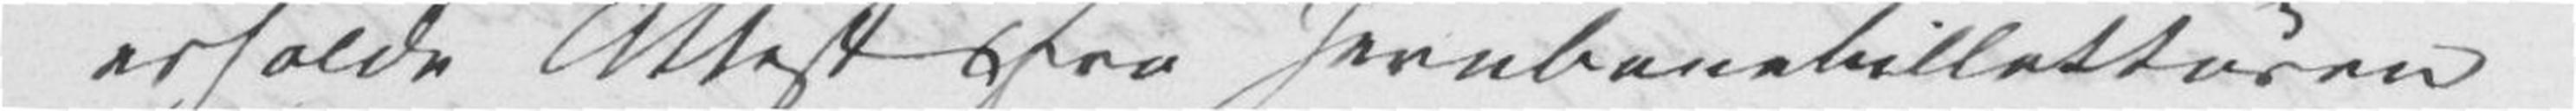erholde Attest fra Jernbanebilletturen
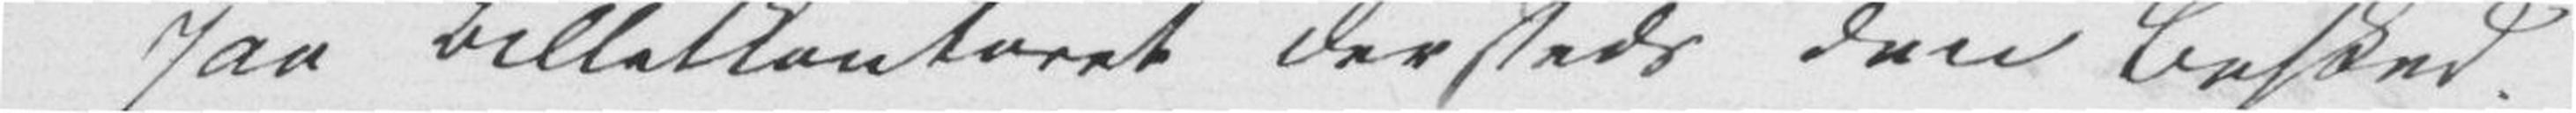paa Billetkontoret dersteds den Besked
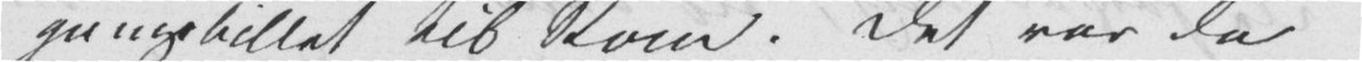gangsbillet til Rom. Det var da
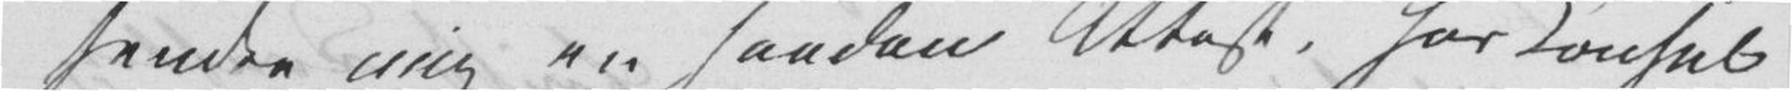sende mig en saadan Attest, har Konsul
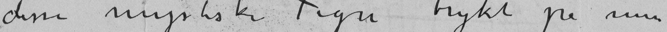disse mystiske Tegn trykt på min
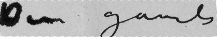Din gamle
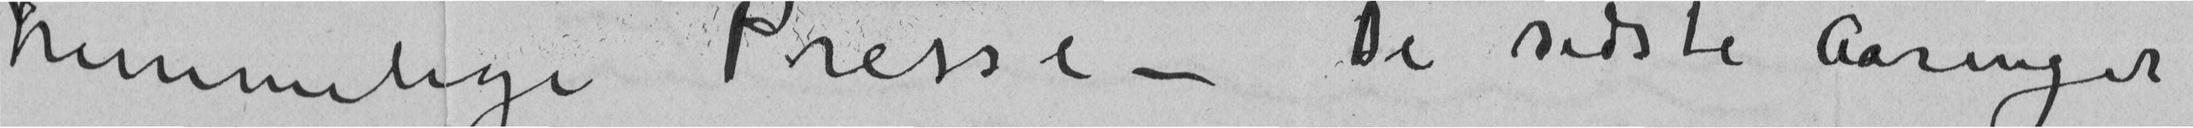hemmelige Presse – De sidste Aaringer
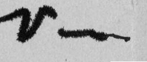Ven
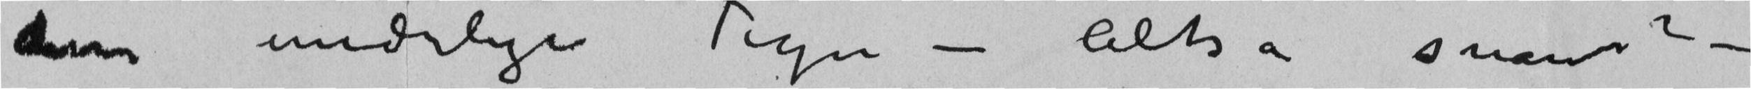disse underlige Tegn – altsaa snarest? –
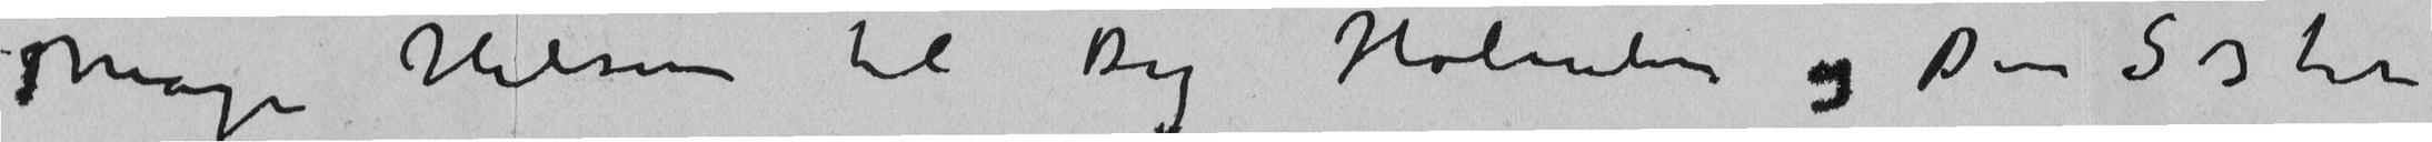Mange hilsener til Dig Holmboe og Din Søster
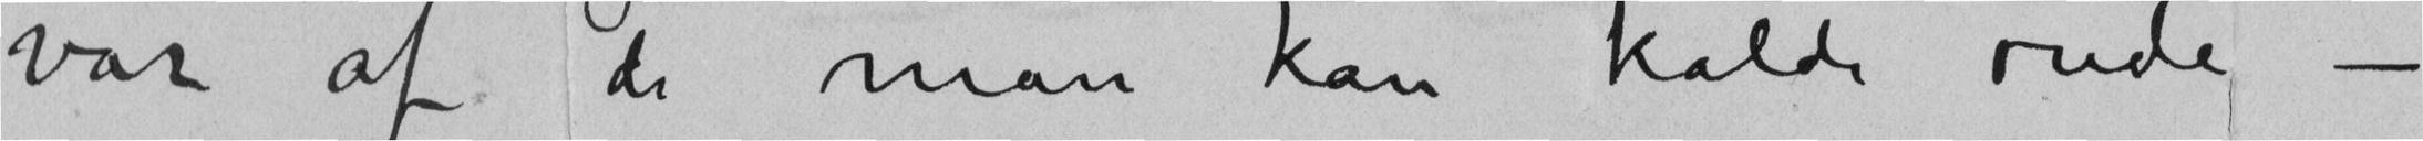var af de man kalde onde –
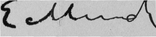E Munch
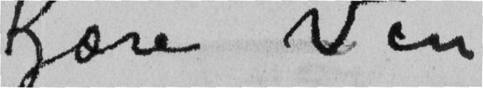Kjære Ven
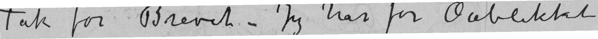Tak for Brevet – Jeg har for Øieblikket
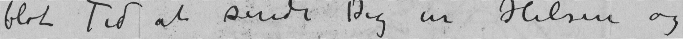blot Tid at sende Dig en Hilsen og
